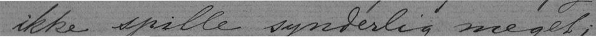ikke spille synderlig meget;
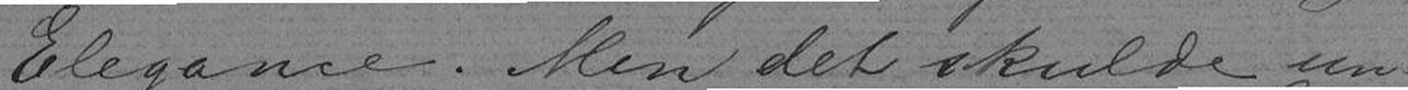Elegance. Men det skulde un-
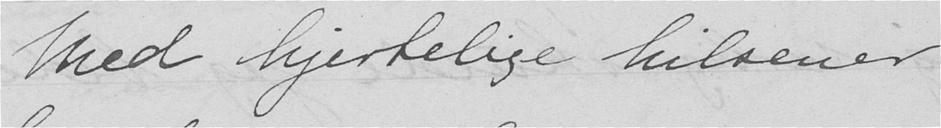Med hjertelige hilsener
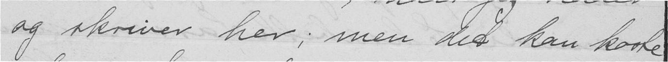og skriver her; men det kan koste
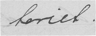toriet.
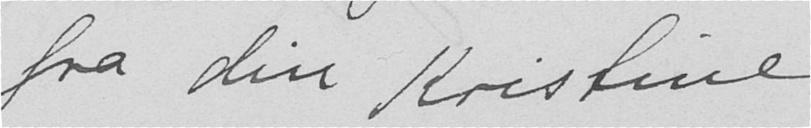fra din Kristine.
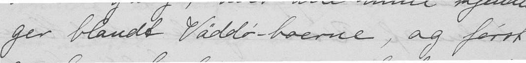ger blandt Väddø-boerne, og først
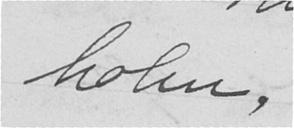holm.
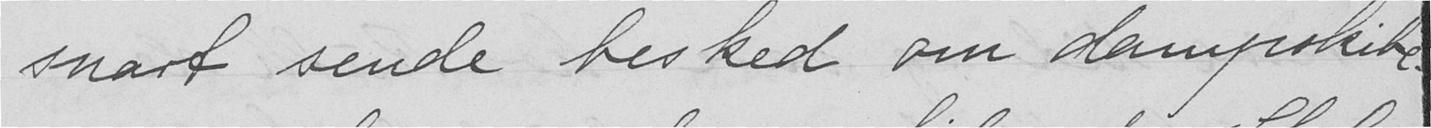snart sende besked om dampskibe-
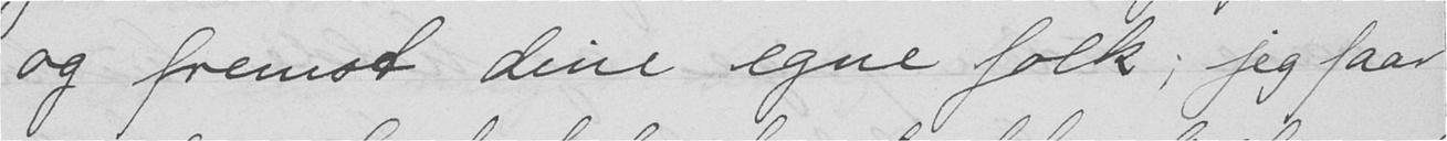og fremst dine egne folk; jeg faar
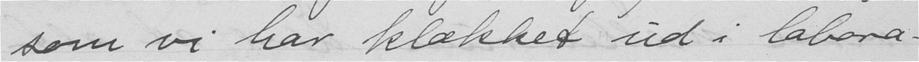som vi har klækket ud i labora-
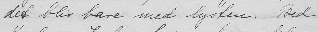det blir bare med lysten. Bed
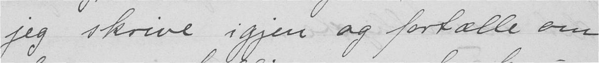jeg skrive igjen og fortælle om
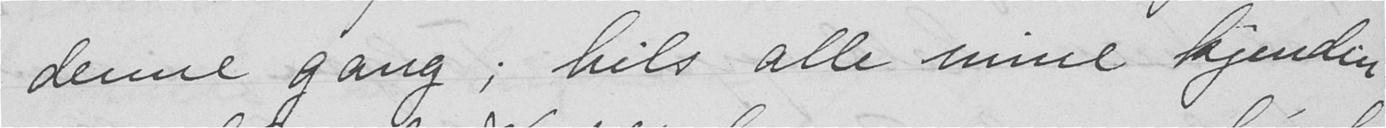denne gang; hils alle mine kjendin-
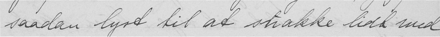saadan lyst til at snakke lidt med
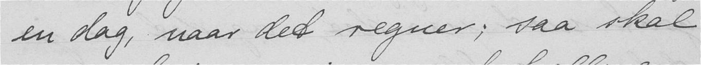en dag, naar det regner; saa skal
